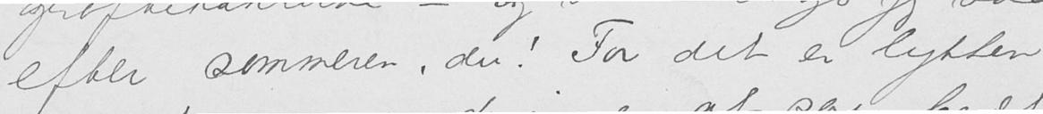efter sommeren, du! For det er lykken
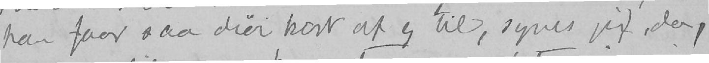han faar saa drøi kost af og til, synes jeg, da,
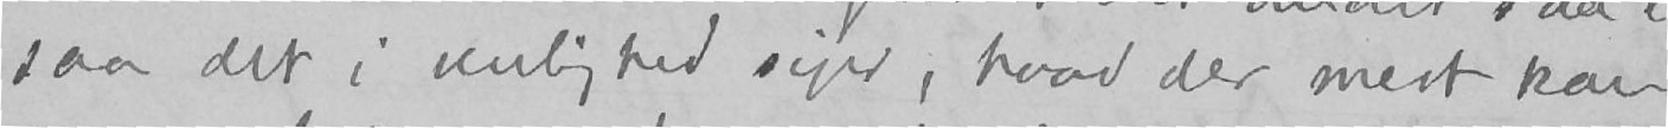saa det i venlighed siger, hvad der mest kan
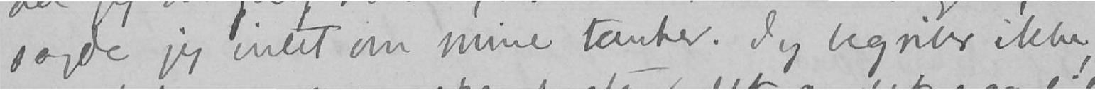sagde jeg intet om mine tanker. Jeg begriber ikke,
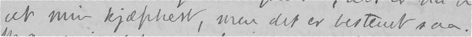vet min kjæphest, men det er bestemt saa.
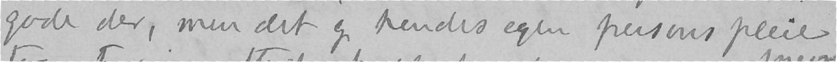gode der, men det og hendes egen persons pleie
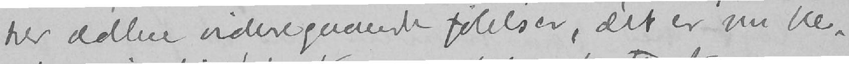ker ædlere videregaaende følelser, det er nu ble-
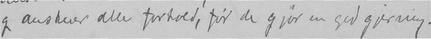og anskuer alle forhold, før de gjør en god gjerning.
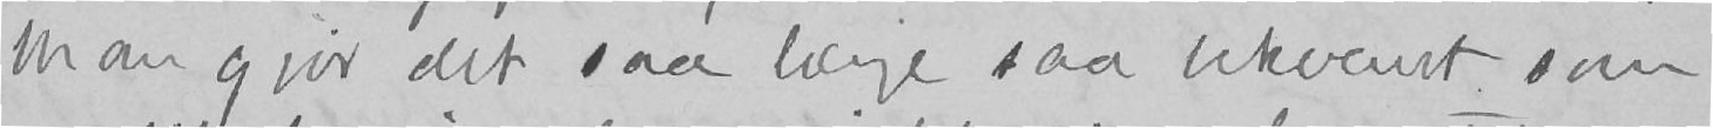Man gjør det saa længe saa bekvemt som
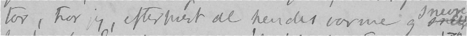tar, tror jeg, efterhvert al hendes varme og snevrer
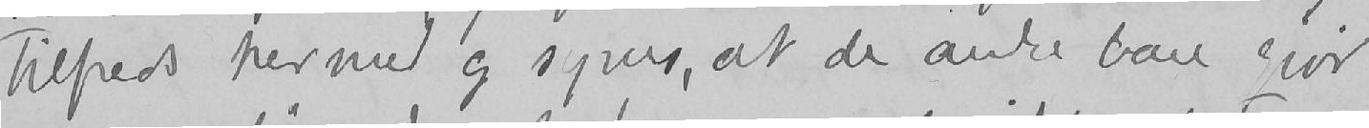tilfreds hermed og synes, at de andre bare gjør
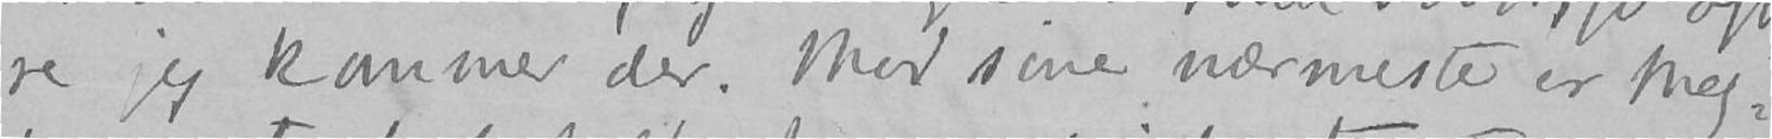re jeg kommer der. Med sine nærmeste er Mag-
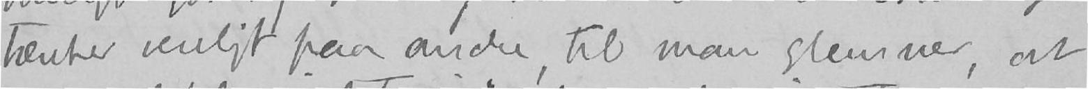tænker venligt paa andre, til man glemmer, at
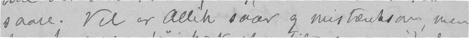saare. Vel er Allik saar og mistænksom, men
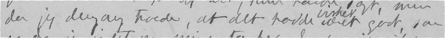da jeg dengang troede, at det havde været godt, saa
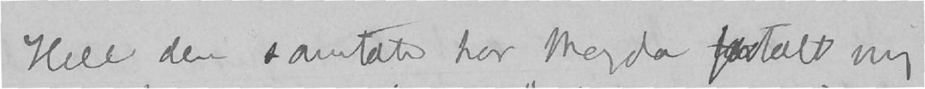Hele den samtale har Magda fortalt mig
